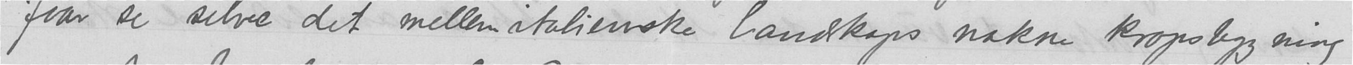faar se selve det mellemitalienske landskaps nakne kropsbygning
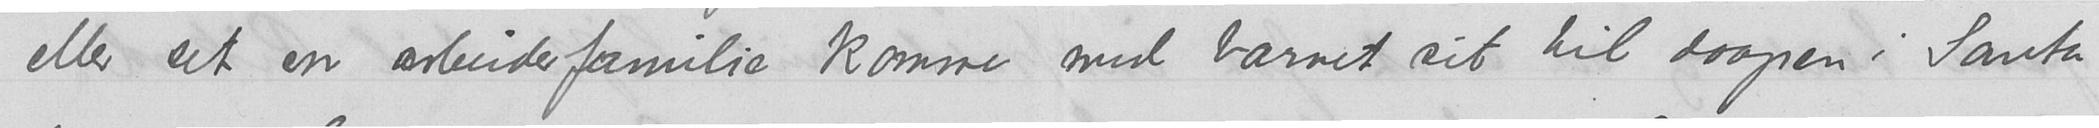eller set en arbeiderfamilie komme med barnet sit til daapen i Santa
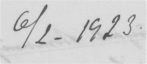6/2-1923.
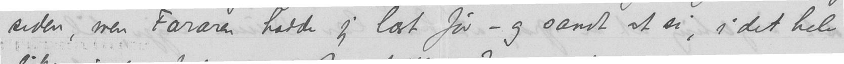siden, men Fararen hadde jeg læst før – og sandt at si, i det hele
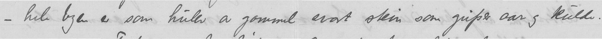- hele byen er som huler av gammel svært stein som gufser aar og kulde.
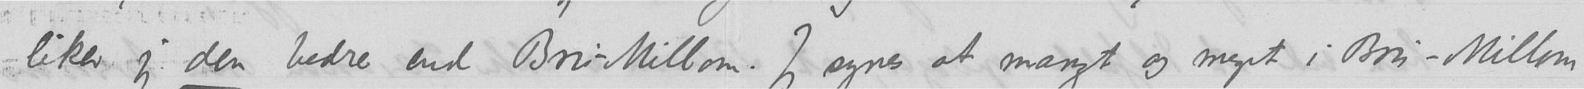liker jeg den bedre end Bru-Millom. Jeg synes at mangt og meget i Bru-Millom
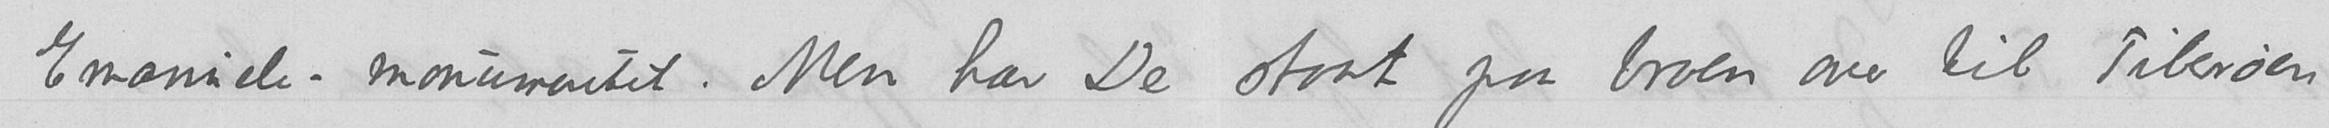Emanuele-monumentet. Men har De staat paa broen over til Tiberøen
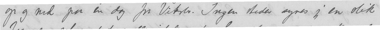op og ned paa en dag fra Viterbo. Ingen steder synes jeg en slik
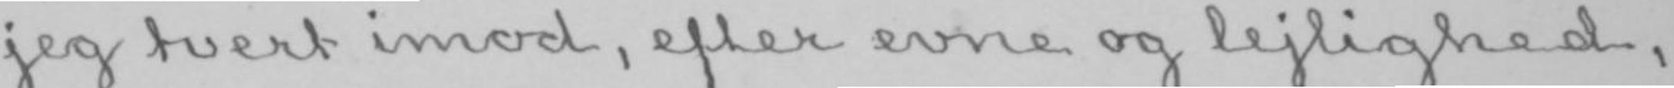jeg tvert imod, efter evne og lejlighed,
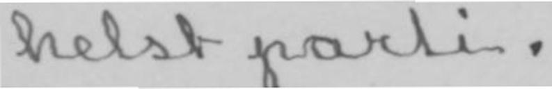helst parti.
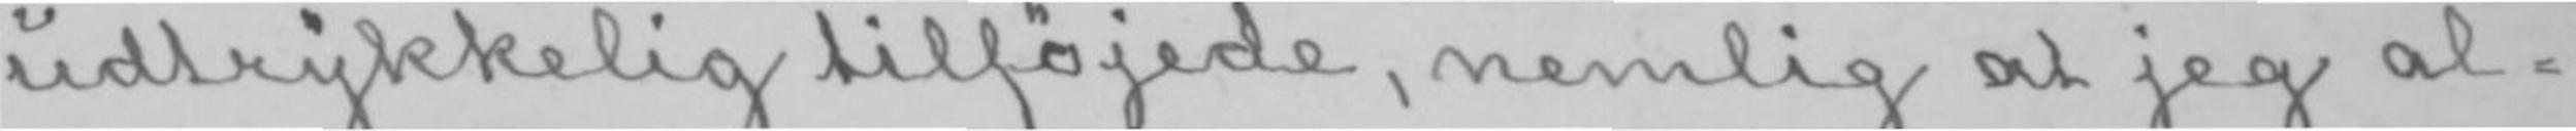udtrykkelig tilføjede, nemlig at jeg al-
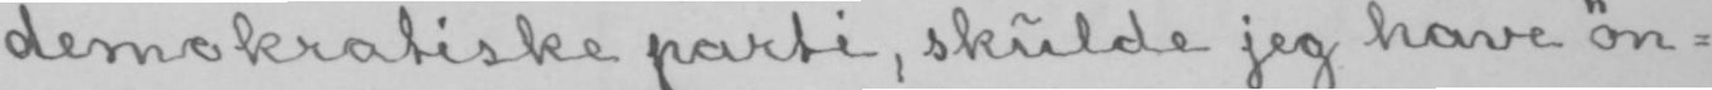demokratiske parti, skulde jeg have øn-
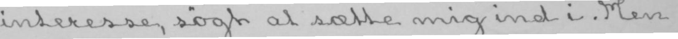interesse, søgt at sætte mig ind i. Men
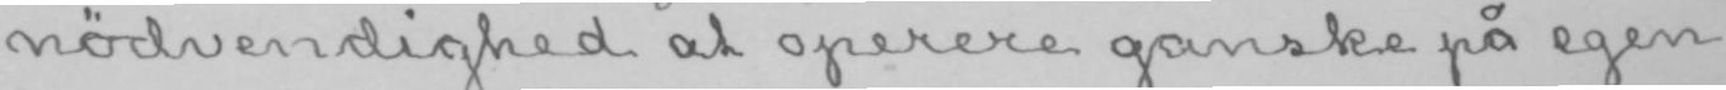nødvendighed at operere ganske på egen
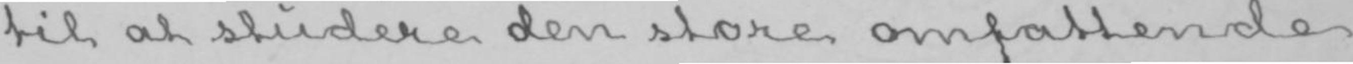til at studere den store omfattende
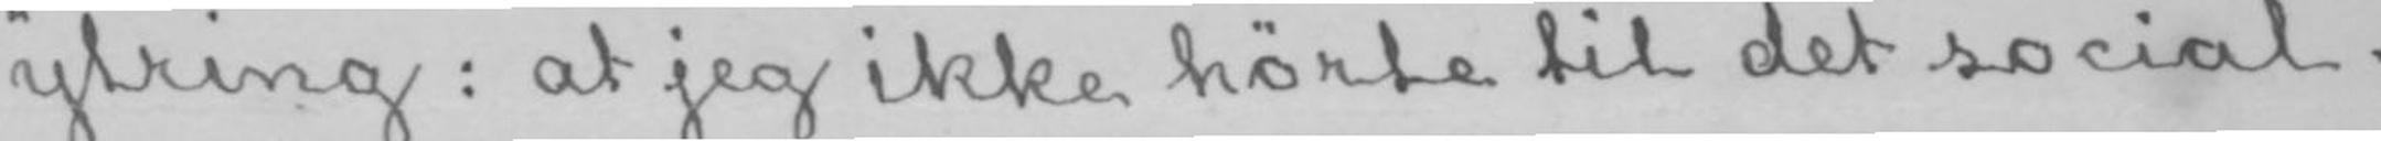ytring: at jeg ikke hørte til det social-
